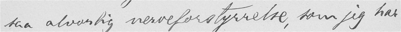saa alvorlig nerveforstyrrelse, som jeg har
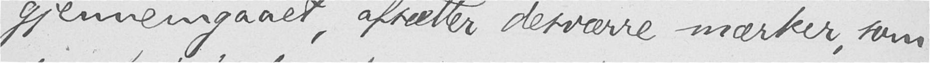gjennemgaaet, afsætter desværre mærker, som
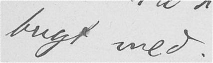bugt med.
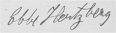Ebbe Hertzberg.
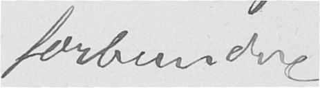forbundne
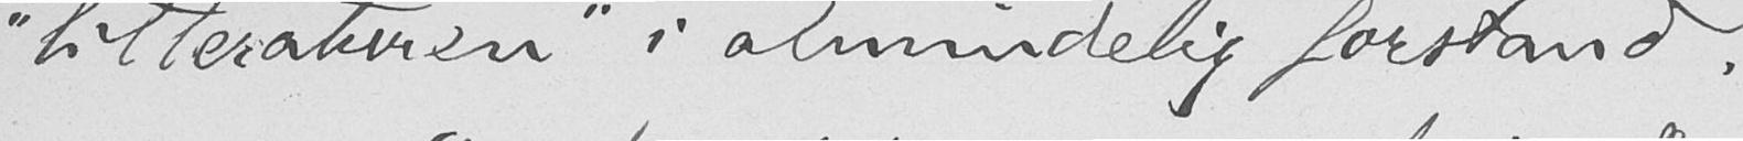"litteraturen" i almindelig forstand.
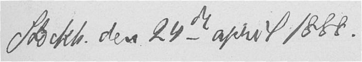Stockh. den 24de april 1888.
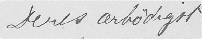Deres ærbødigst
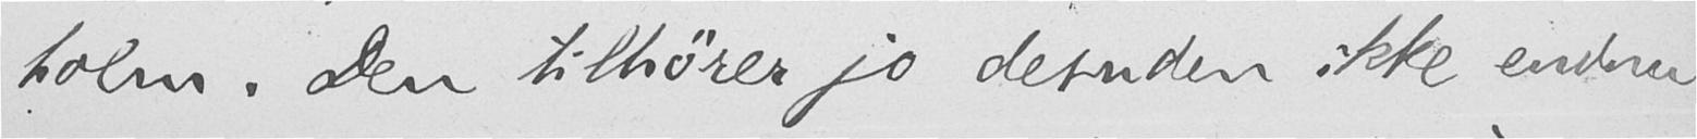holm. Den tilhører jo desuden ikke endnu
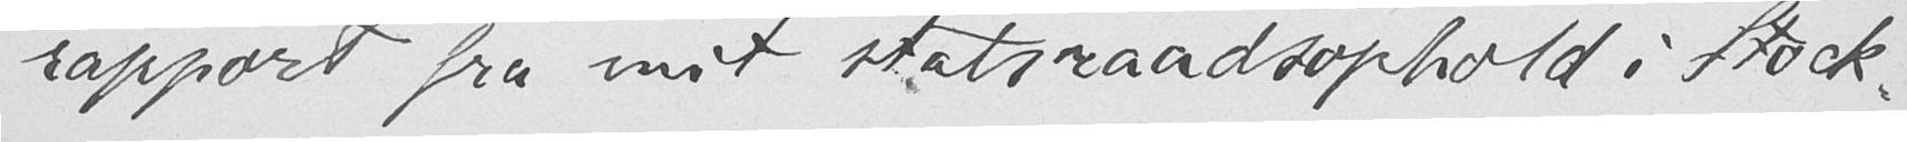rapport fra mit statsraadsophold i Stock-
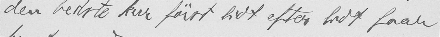den bedste kur først lidt efter lidt faar
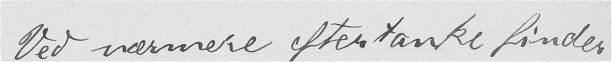Ved nærmere eftertanke finder
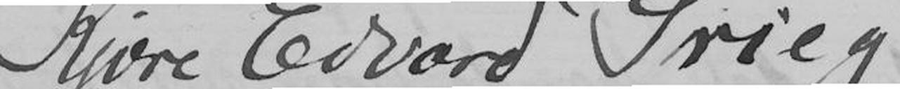Kjære Edvard Grieg!
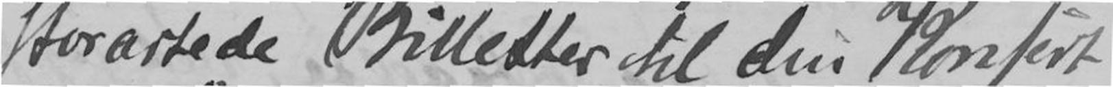storartede Billetter til din Konsert
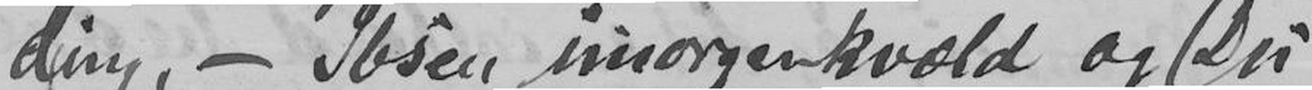ding, - Ibsen imorgenkvæld og Du
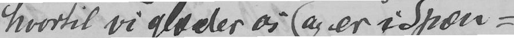hvortil vi glæder os og er i Spæn-
